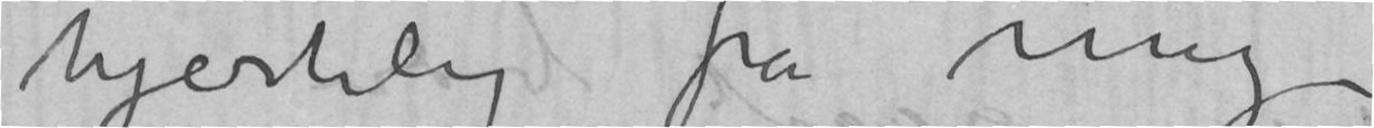hjertelig fra mig
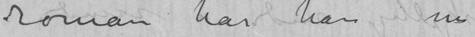roman har han nu
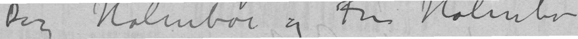Dig Holmboe og Fru Holmboe
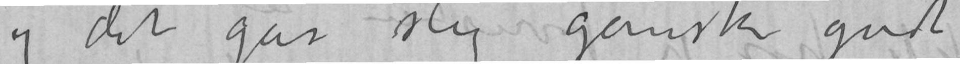og det går slig ganske godt
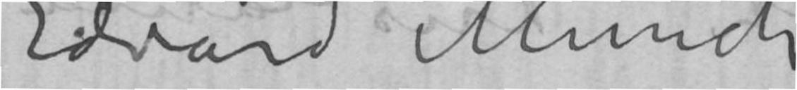Edvard Munch
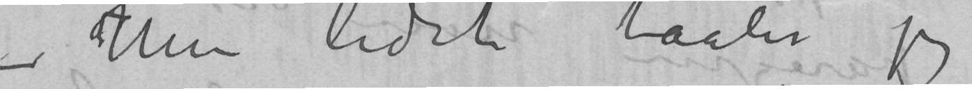– Men lidet taaler jeg
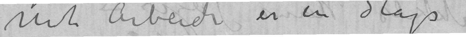Mit Arbeide er en Slags
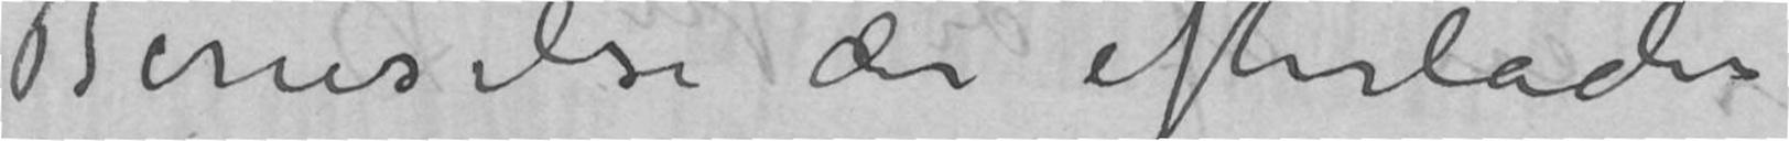Beruselse der efterlader
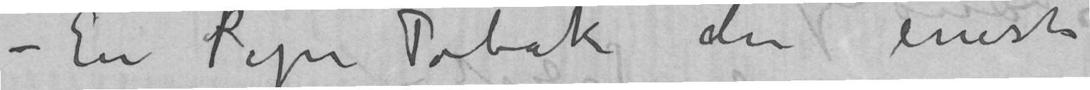–	En Pipe Tobak den eneste
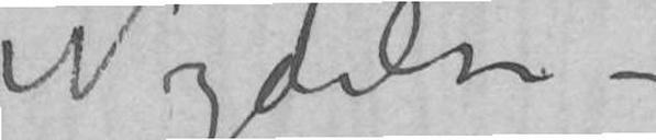Nydelse –
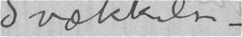Svækkelse –
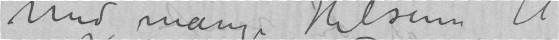Med mange Hilsener til
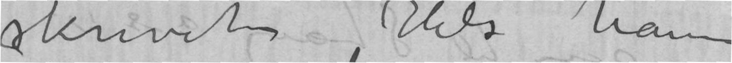skrevetHils ham
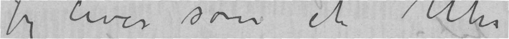Jeg lever som et Uhr
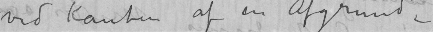ved Kanten af en Afgrund –
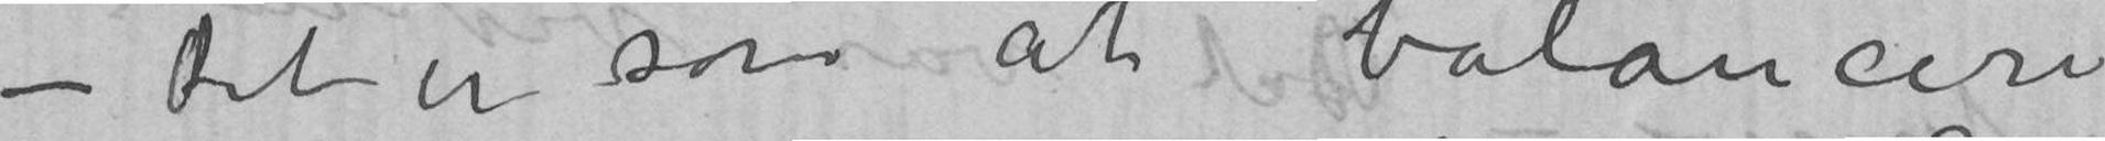– Det er som at balancere
